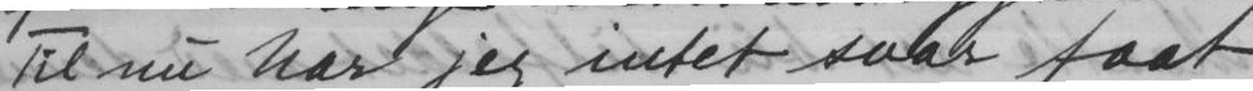Til nu har jeg intet svar faat.
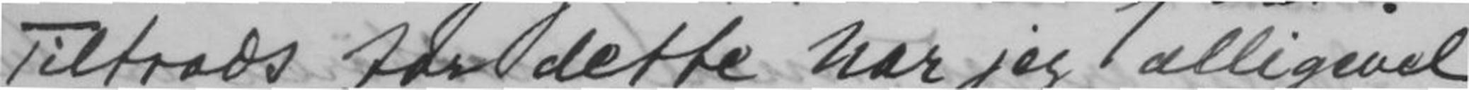Tiltrods for dette har jeg alligevel
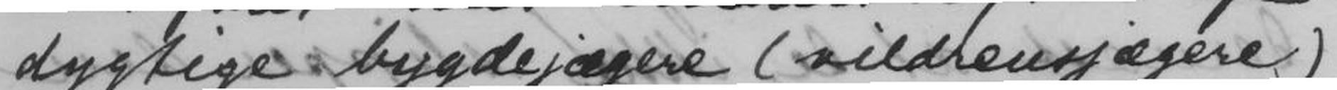dygtige bygdejægere (vildreinsjægere)
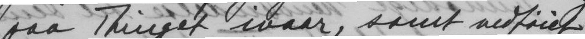påå Thinget ivaar, samt vedføiet
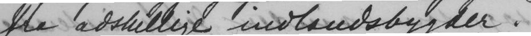fra adskillige indlandsbygder.
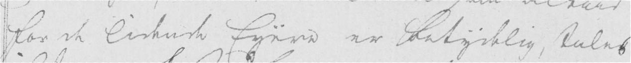for de lidende Eyere er betydelig, tales
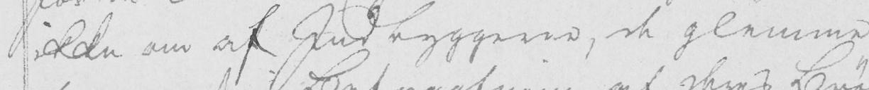ikke om af Indbyggerne, de glemme
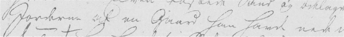Jorderne af en Gaard han havde nede i
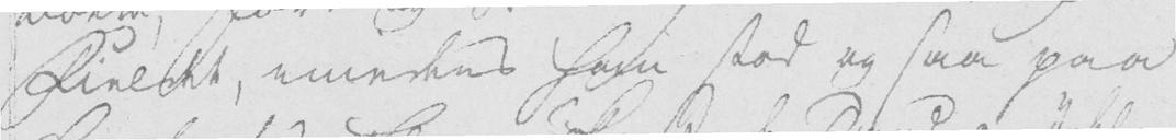Fieldet, imedens han stod og saa paa
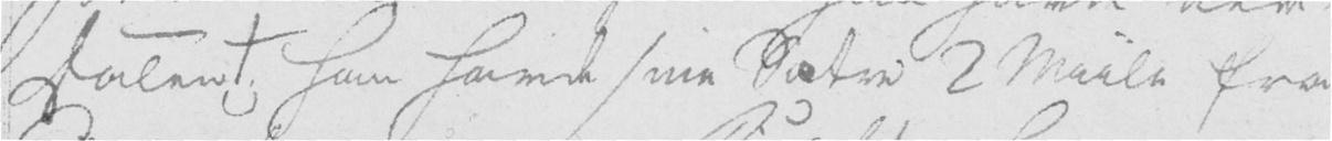Dalen +; han havde sine Sætre 2 Miile fra
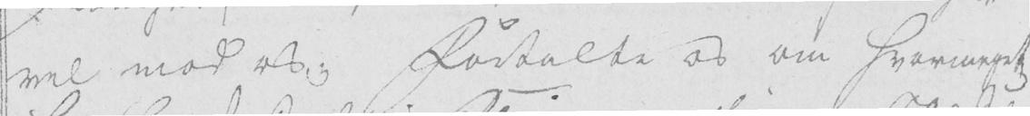vel mod os; Fortalte os om hvormeget
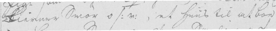kierner Smør o s. v., et Huus til at boe
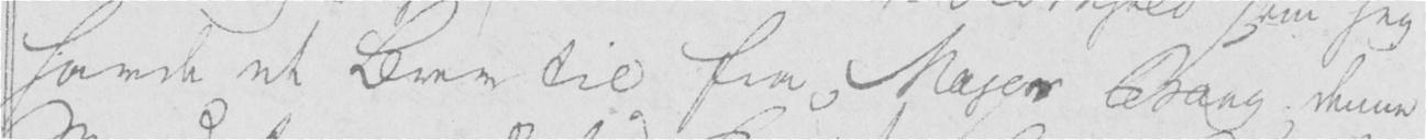havde et Brev til fra Major Bang; denne
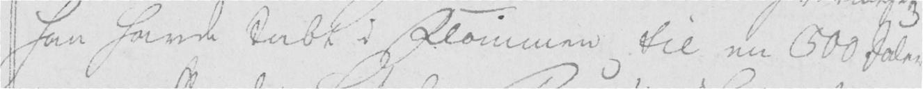han havde tabt i Flommen til en 500 Daler
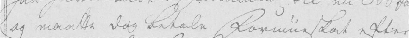og maatte dog betale Formueskat efter
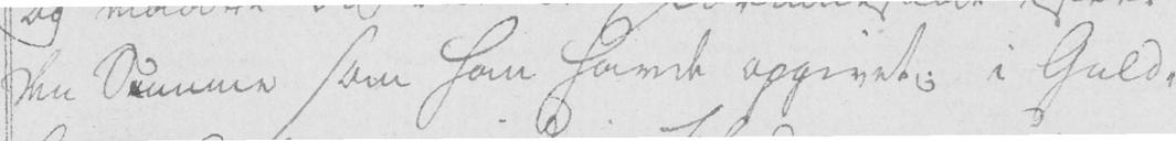den Summen som han havde opgivet; i Guld-
